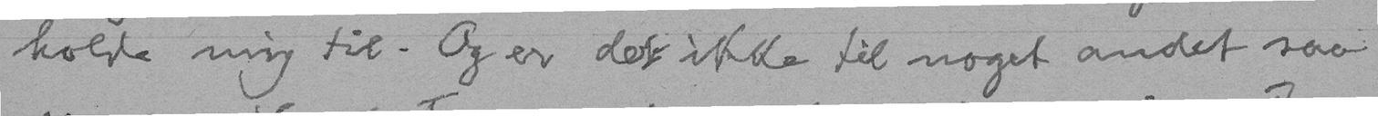holde mig til. Og er det ikke til noget andet saa
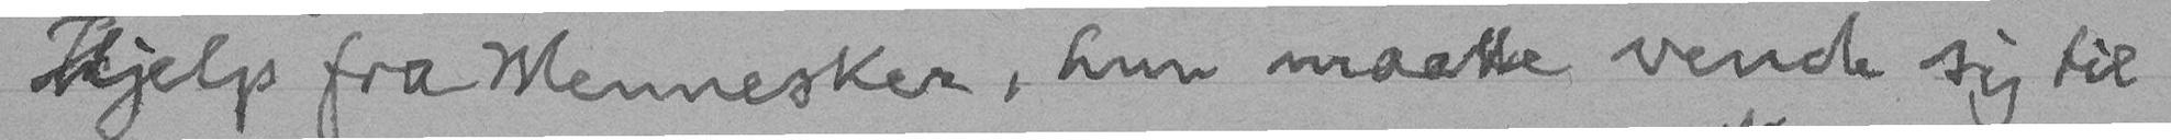Hjelp fra Mennesker, hun maatte vende sig til
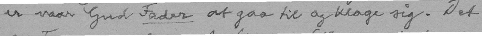er vaar Gud Fader at gaa til og klage sig. Det
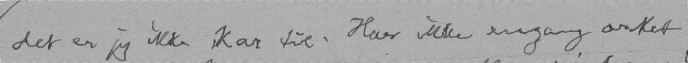det er jeg ikke Kar til. Har ikke engang orket
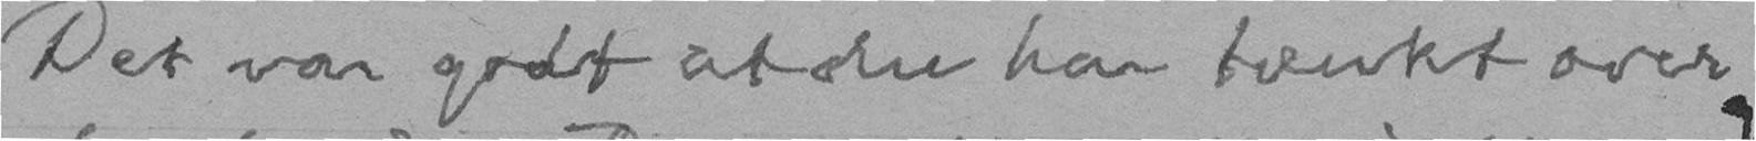Det var godt at du har tænkt over
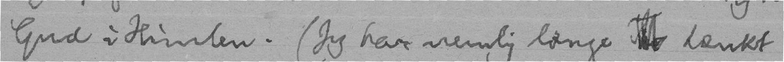Gud i Himlen. (Jeg har nemlig længe  tænkt
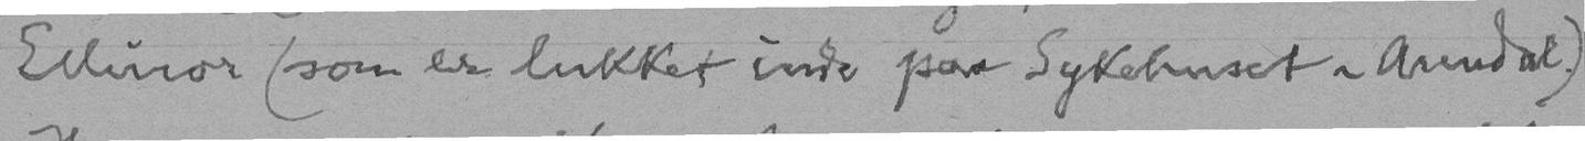Ellinor (som er lukket inde paa Sykehuset i Arendal.)
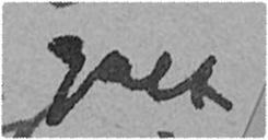galt
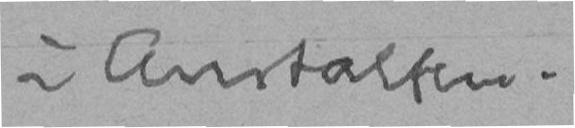i Anstalten.
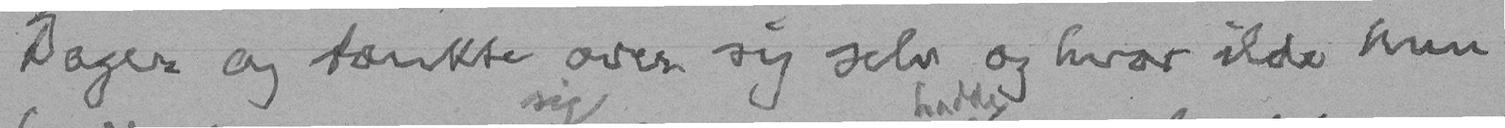Dager og tænkte over sig selv og hvor ilde hun
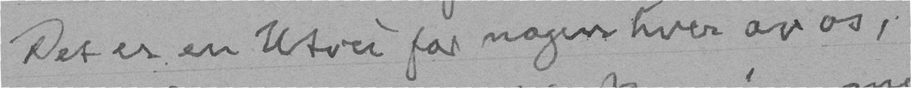Det er en Utvei for nogen hver av os,
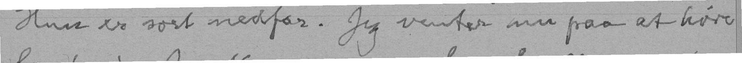Hun er sort nedfor. Jeg venter nu paa at høre
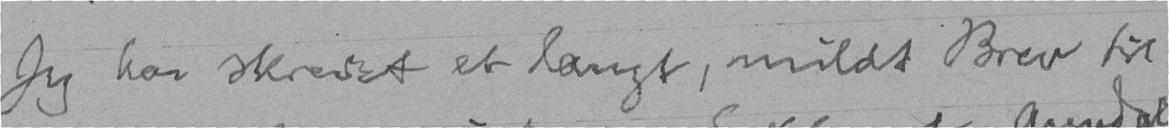Jeg har skrevet et langt, mildt Brev til
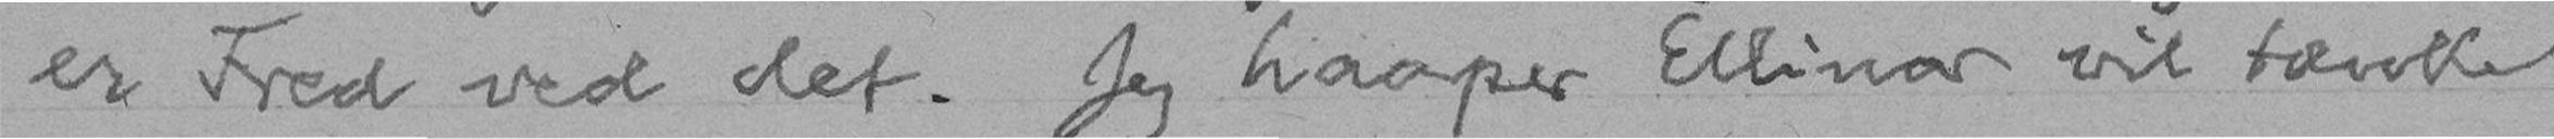er Fred ved det. Jeg haaper Ellinor vil tænke
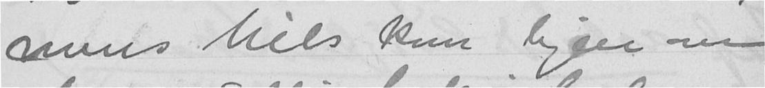mens Nils kom hjem om
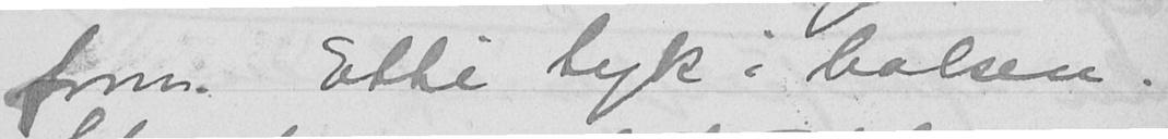form. Etti tyk i halsen.
